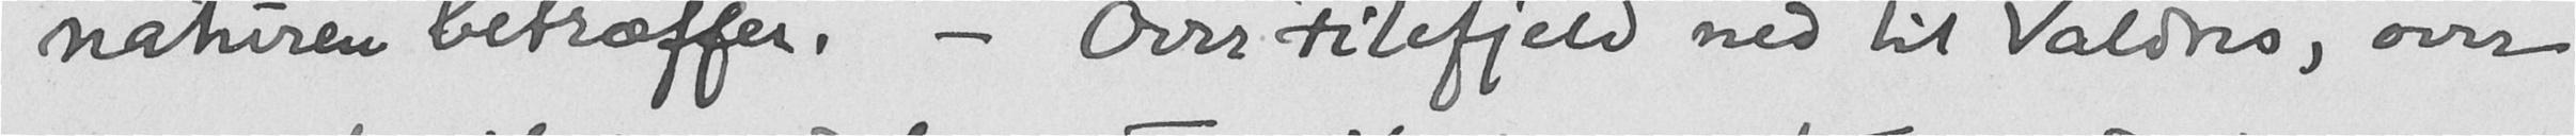naturen betræffer. - Over Filefjeld ned til Valdres, over
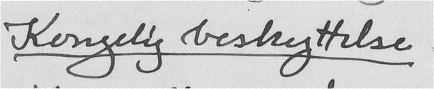Kongelig beskyttelse.
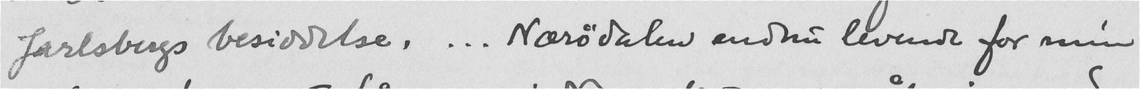Jarlsbergs besiddelse, ... Nærødalen endnu levende for min
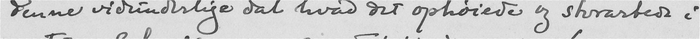denne vidunderlige dal hvad det ophøiede og storartede i
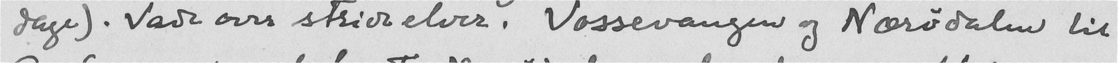dage). Vade over stridselver. Vossevangen og Nærødalen til
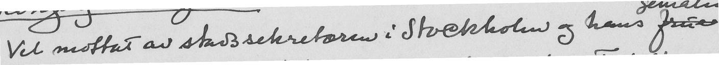Vel mottat av stadssekretæren i Stockholm og hans frue
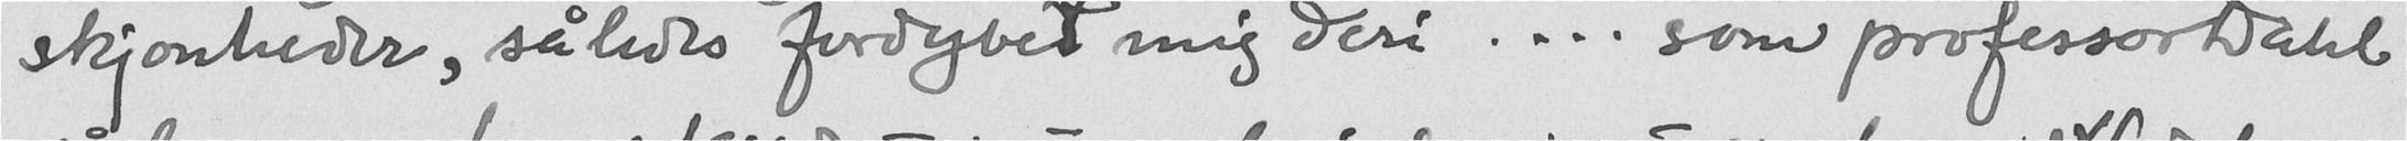skjønheder, således fordybet mig deri.... som professor Dahl
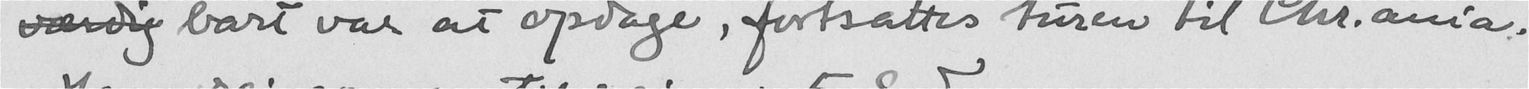værdig bart var at opdage, fortsattes turen til Chr.ania.
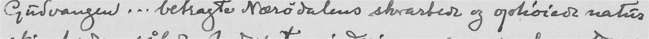Gudvangen... betragte Nærødalens storartede og ophøiede natur
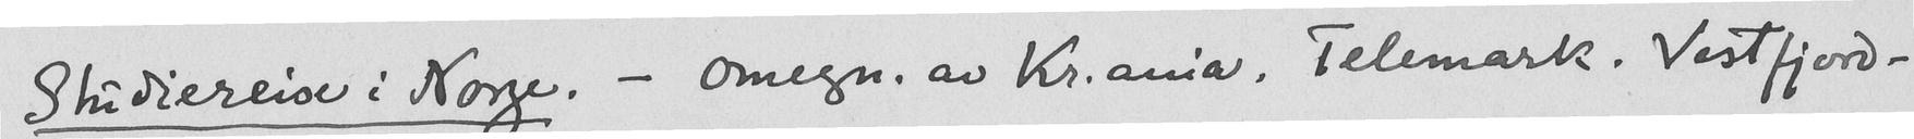Studiereise i Norge. - Omegn. av Kr.ania, Telemark. Vestfjord-
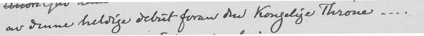av denne heldige debut foran den Kongelige Throne....
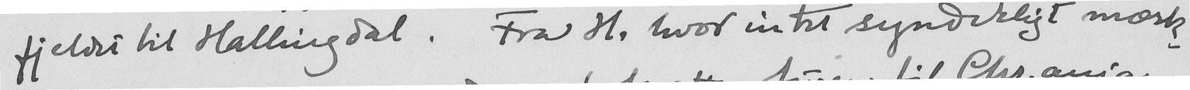fjeldet til Hallingdal. Fra H. hvor intet synderligt mærk-
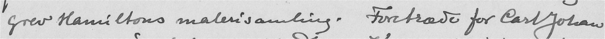Grev Hamiltons malerisamling. Foretræde for Carl Johan
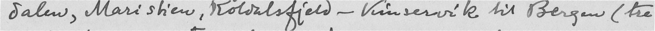dalen, Maristien, Røldalsfjeld - Kinservik til Bergen (tre
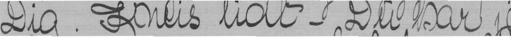Dig. Kneis lidt - Du har jo
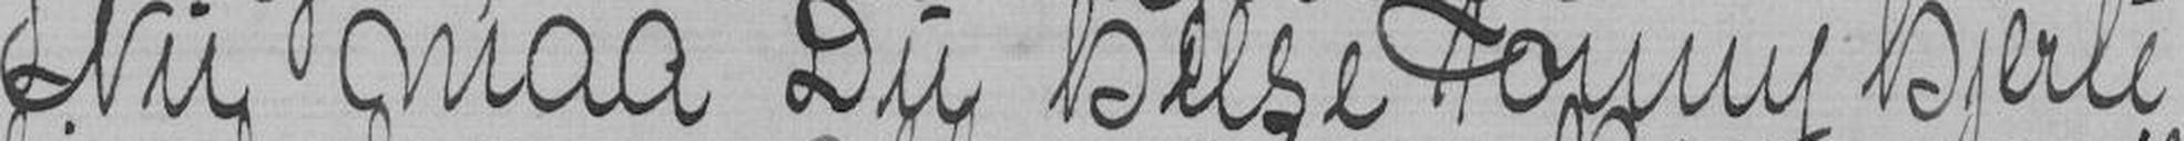Nu maa Du hilse Tonny hjerte
